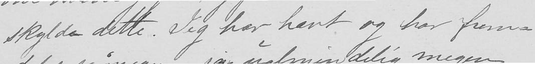skylde dette. Jeg har havt og har frem-
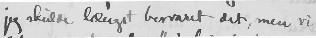jeg skulde længst besvaret det, men vi
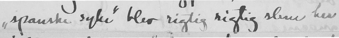"spanske syke" blev rigtig rigtig slem her
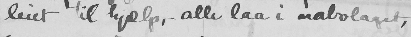leiet til hjælp, - alle laa i nabolaget,
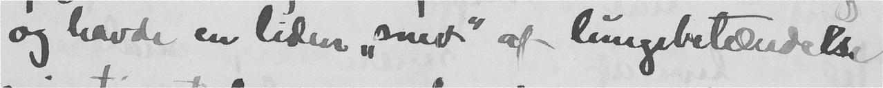og havde en liden "snev" af lungebetændelse
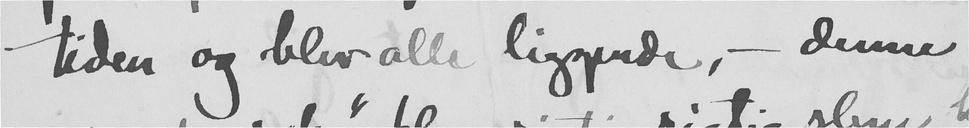tiden og blev alle liggende, - denne
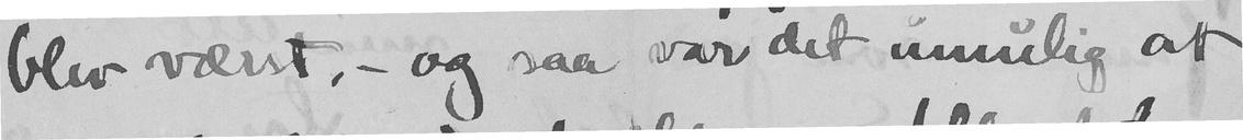blev værst, - og saa var det umulig at
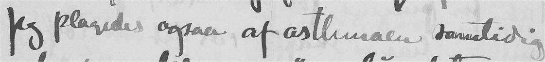Jeg plagedes ogsaa af asthmaen samtidig
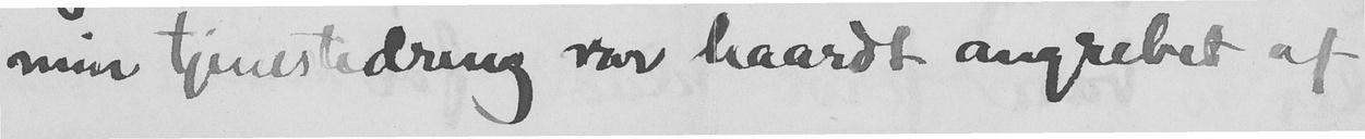min tjenestedreng var haardt angrebet af
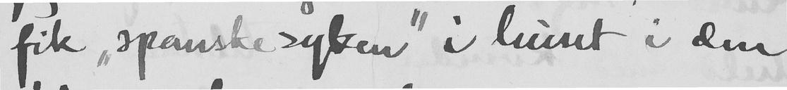fik "spanskesyken" i huset i den
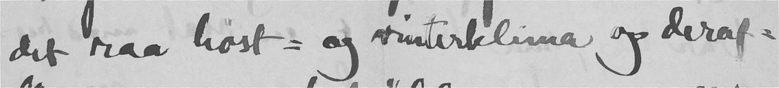det raa høst- og vinterklima og deraf-
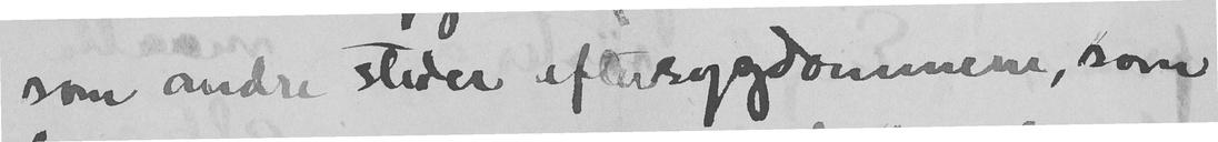som andre steder eftersygdommene, som
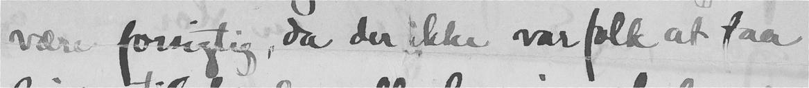være forsigtig, da der ikke var folk at faa
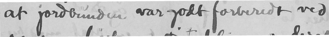at jordbunden var godt forberedt ved
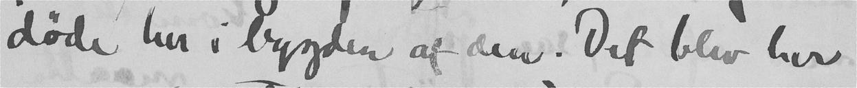døde her i bygden af den. Det blev her
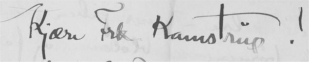Kjære Frk Kamstrup!
